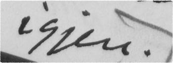igjen.
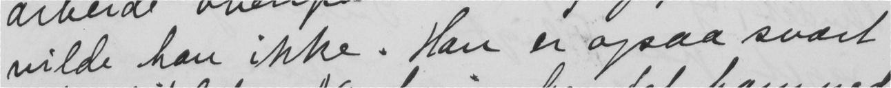vilde han ikke. Han er ogsaa svært
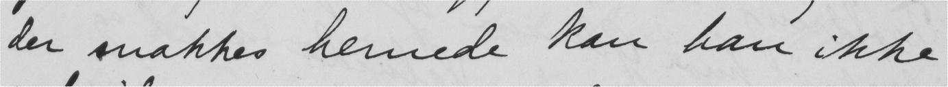der snakkes hernede kan han ikke
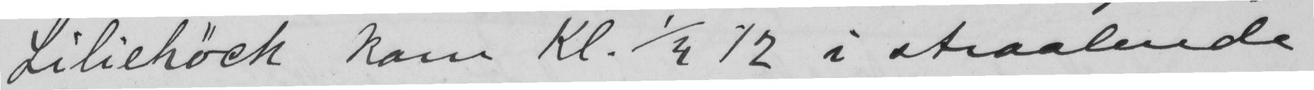Liliehøck kom Kl. 1/4 12 i straalende
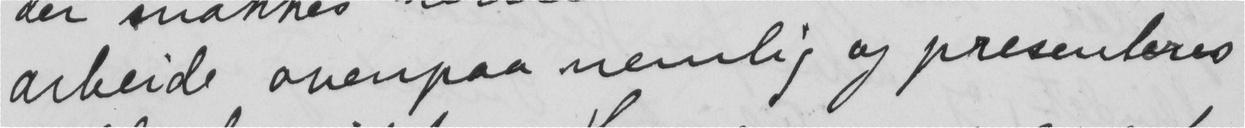arbeide ovenpaa nemlig og presenteres
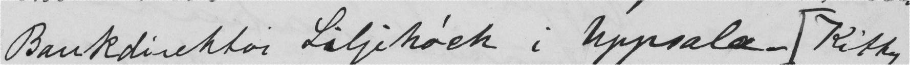Bankdinktør Liljehøck i Uppsala. Kitty
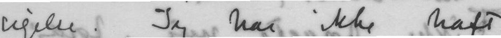sigelser. Jeg har ikke haft
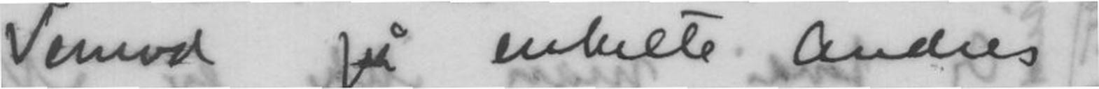Vemod på enkelte Andres
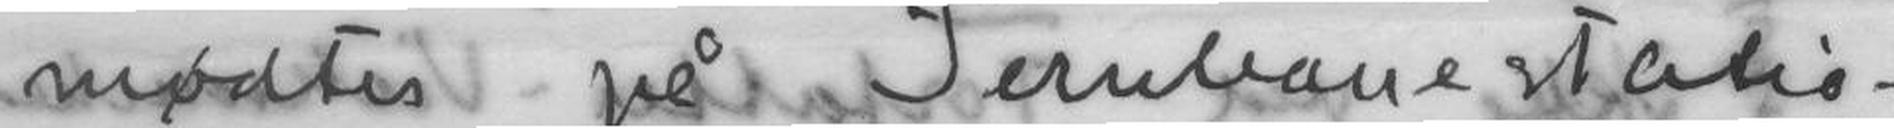mødtes på Jernbane statio-
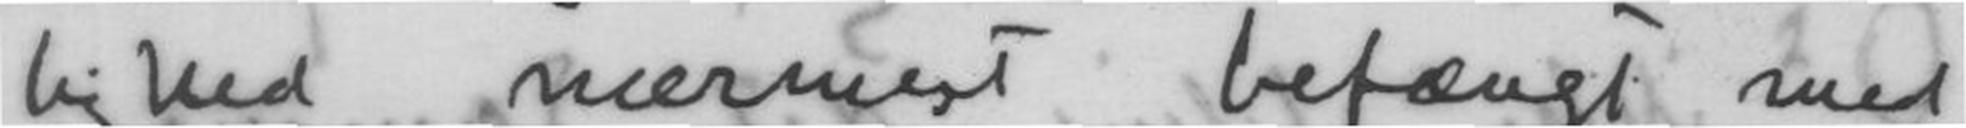lighed nærmest befængt med
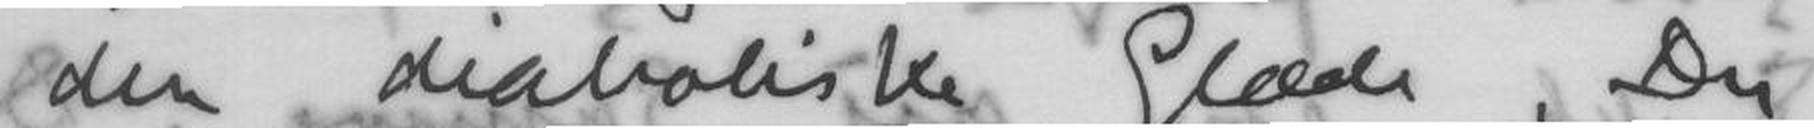den diaboliske Glæde Du
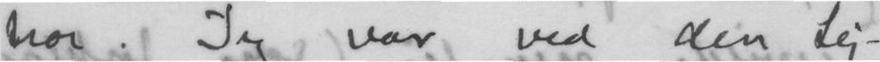tror. Jeg var ved den Lej-
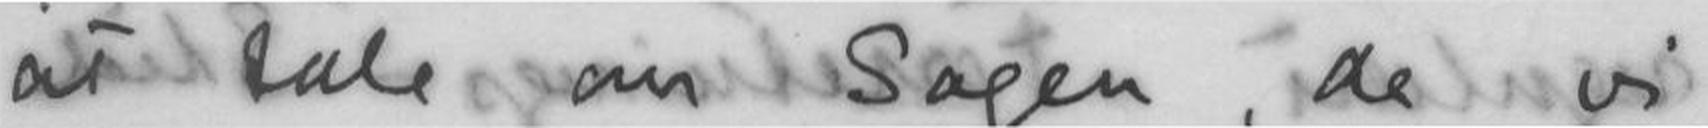at tale om Sagen, de vi
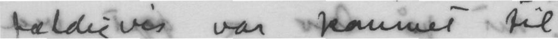fældigvis var hæmmet til
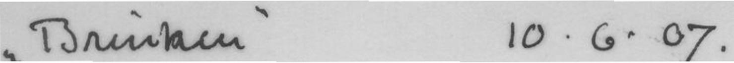Brinken10.6.07
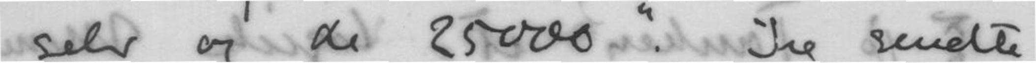selv og de 25000". Jeg sendte
